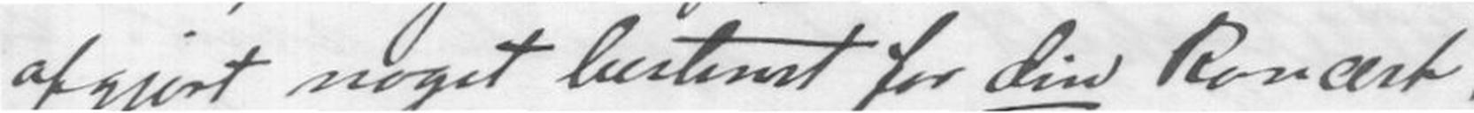afgjort noget bestemt for din koncert,
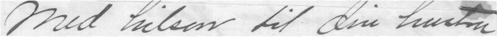Med hilsen til din hustru
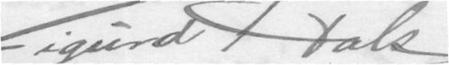Sigurd Hals
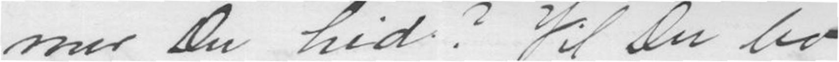mer Du hid? Vil Du bo
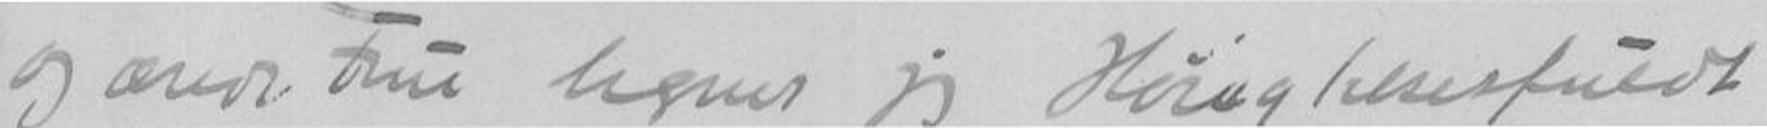og erede Fru tegner jeg Høiagtelsesfuldt
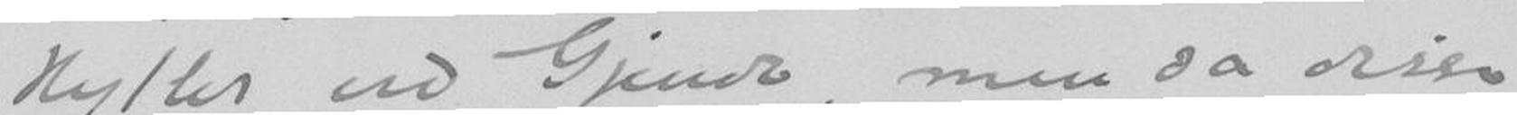Hytter ved Gjende, men da disse
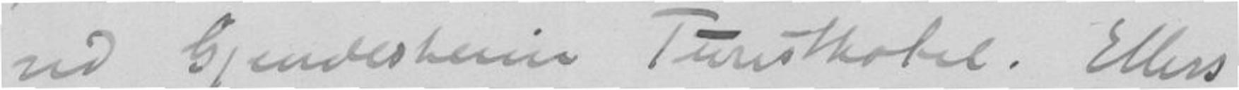ved Gjendesheim Turisthotel. Ellers
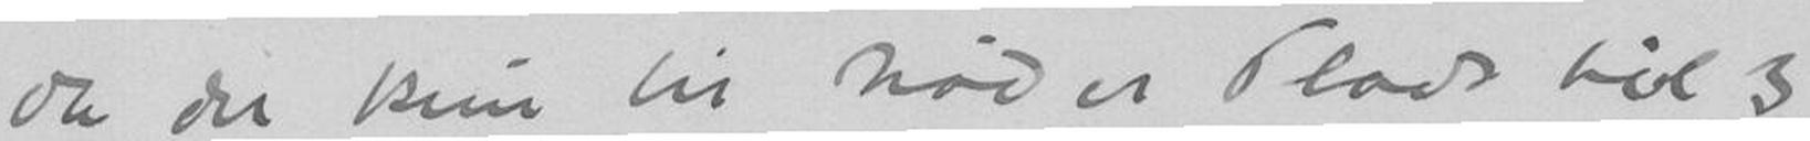der du kun til Nød er Plads til 3
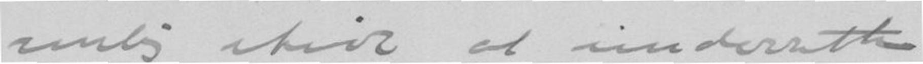venlig itide at underrætte
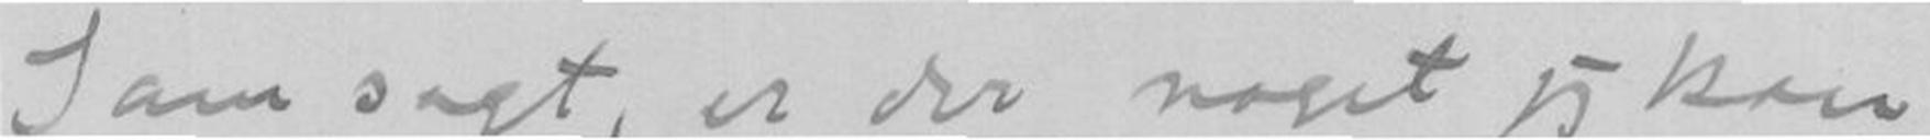Som sagt, er der noget jeg kan
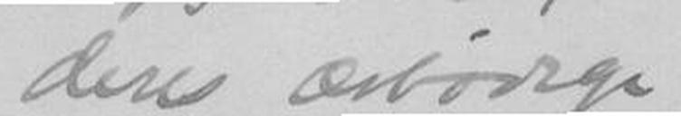deres ærbødige
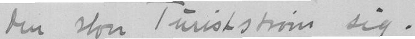ikke den store Turiststrøm sig.
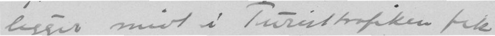ligger midt i Turisttrafiken fik
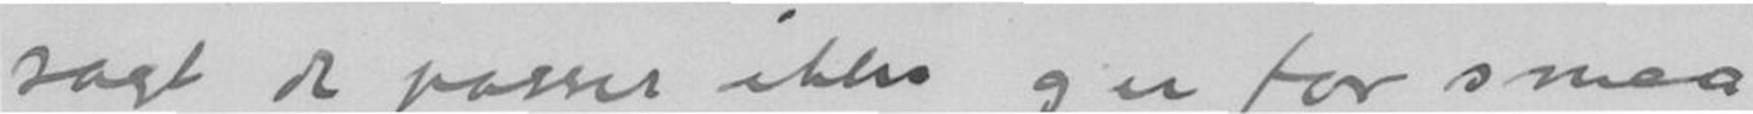sagt de passer ikke og er for smaa
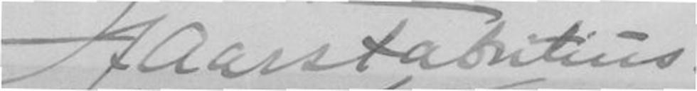J.F. Aars Fabritius.
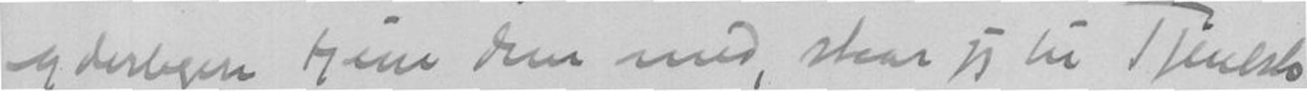yderlegen tjene de, med, staar jeg til Tjeneste.
